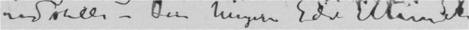udstille – Din hengivne Edv Munch
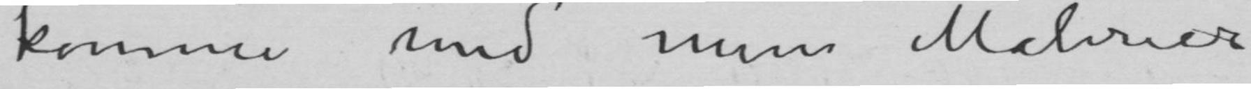komme med mine Malerier
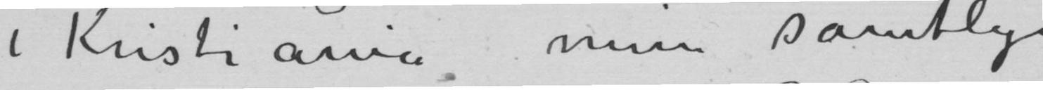i Kristiania mine samtlige
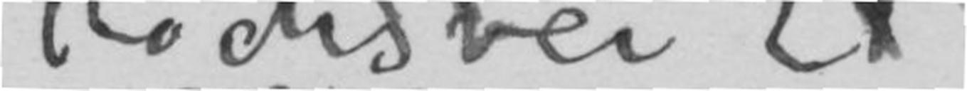Kochsvei 21
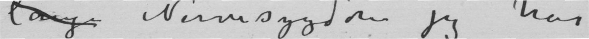lange Nervesygdom jeg har
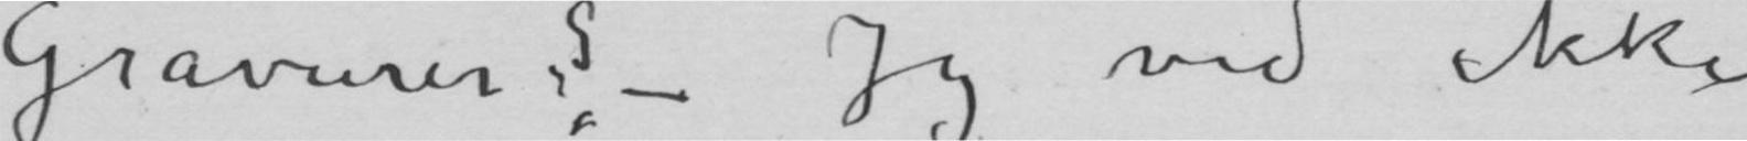Gravurer? – Jeg ved ikke
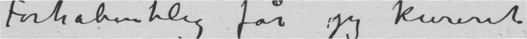Forhåbentlig får jeg kureret
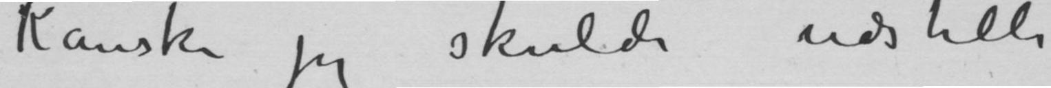Kanske jeg skulde udstille
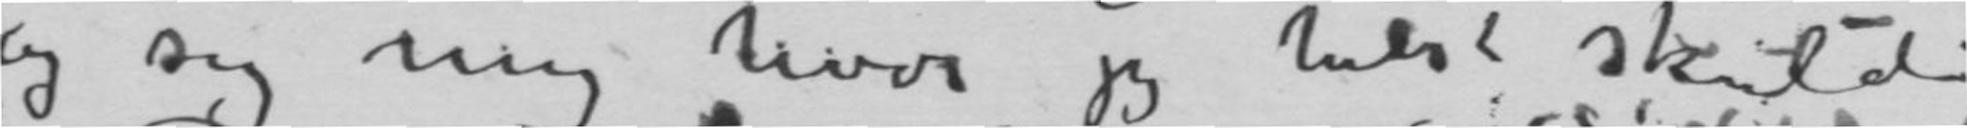og sig mig hvor jeg helst skulde
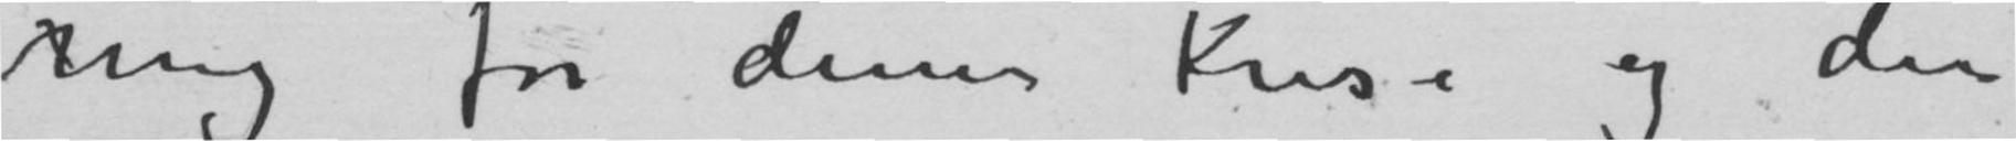mig for denne Krise og den
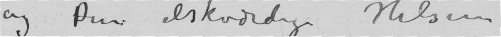og Din elskværdige Hilsen
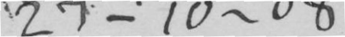27-10-08
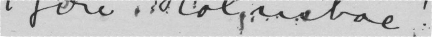Kjære Holmboe!
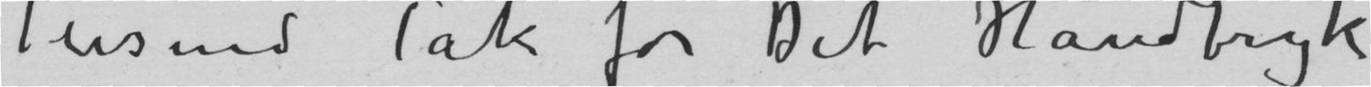Tusind Tak for Det Håndtryk
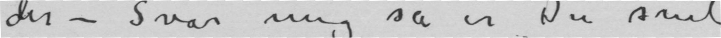der – Svar mig så er Du snil
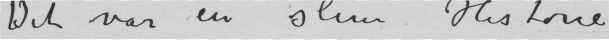Det var en slem Historie
